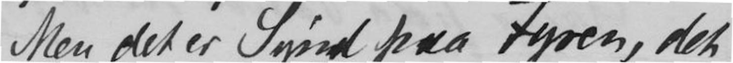Men det er Synd paa Fyren, det
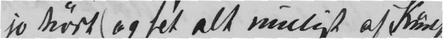jo hørt og sét alt muligt af Kunst
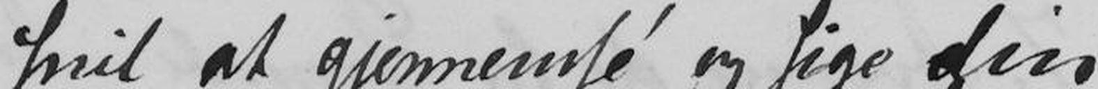snil at gjennemse og sige din
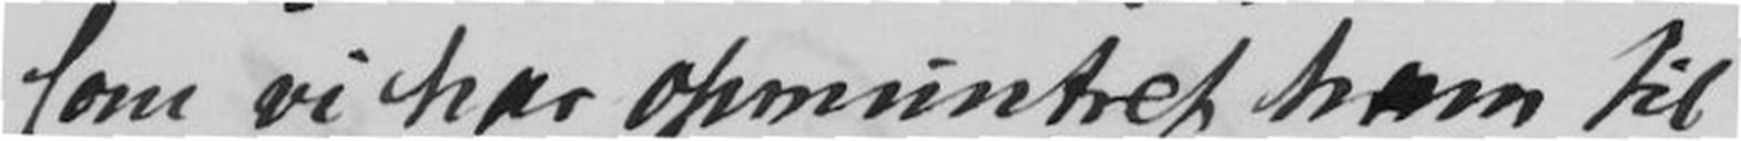som vi har opmuntret ham til
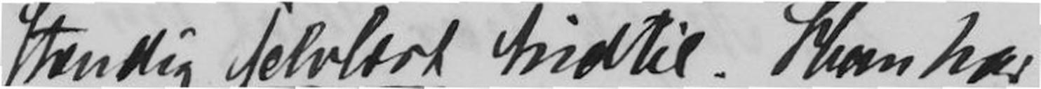stændig selvlært hidtil. Han har
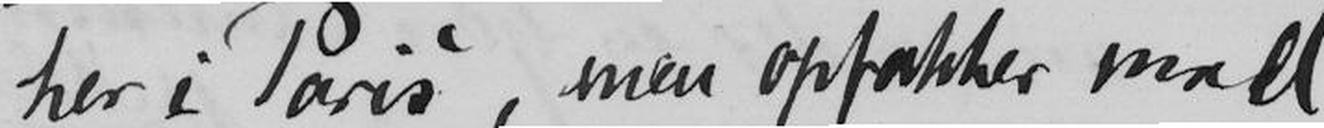her i Paris, men opfatter med
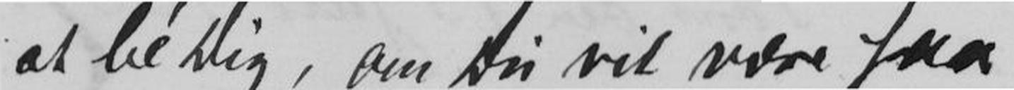at be Dig, om Du vil være saa
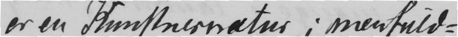er en Kunstnernatur; men fuld-
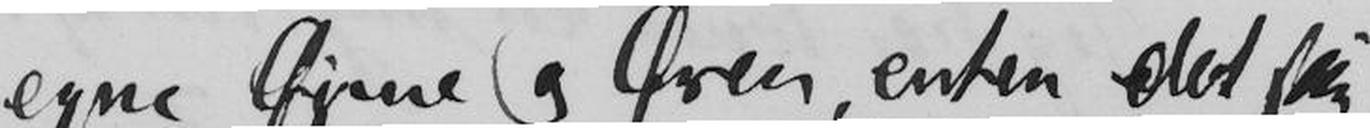egne Øjne og Øren, enten det saa
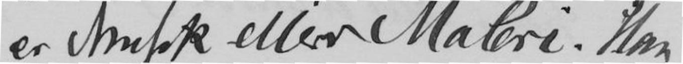er Musik eller Maleri. Han
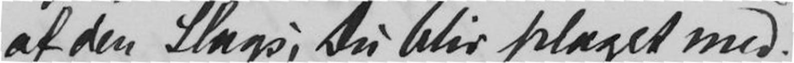af den Slags; Du blir plaget med.
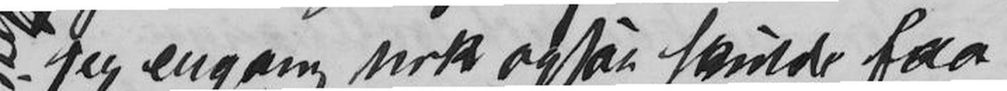jeg engang nok ogsaa skulde faa
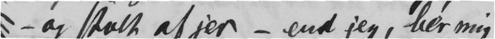- og stolt af jer - end jeg, ber mig
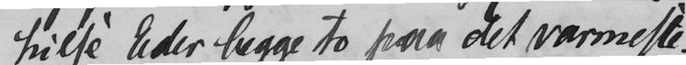hilse Eder begge to paa det varmeste.
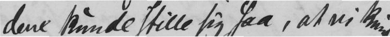dene kunde stille sig saa, at vi kunde
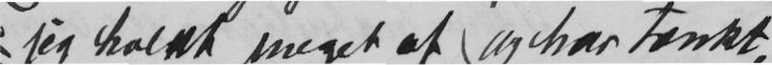jeg holdt meget af og har tænkt,
